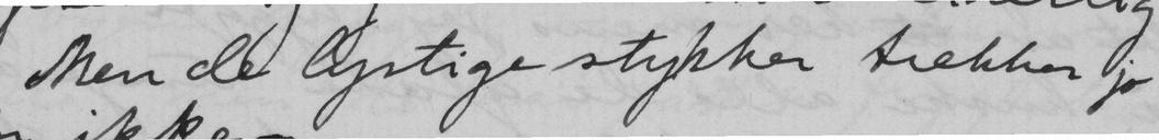Men de lystige stykker trekker jo
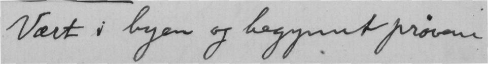Vært i byen og begynnt prøvene
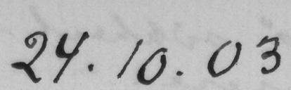24.10.03
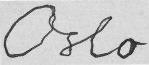Oslo
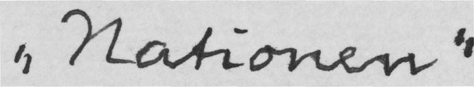"Nationen"
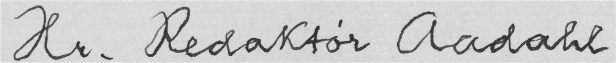Hr. Redaktør Aadahl
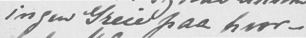ingen Greie paa hvor-
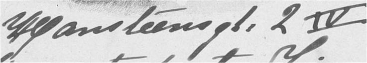Hansteensgt. 2 IV
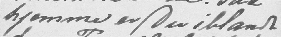hjemme er Du iblandt
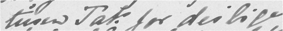tusen Tak for deilige
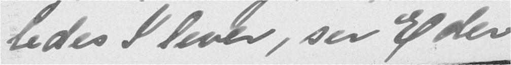ledes I lever, ser Eder
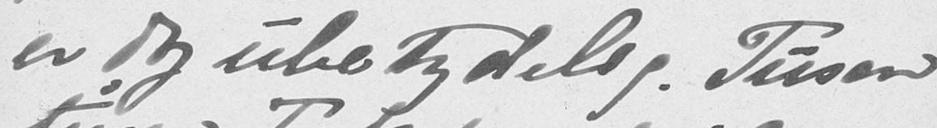er dog ubetydelig. Tusen
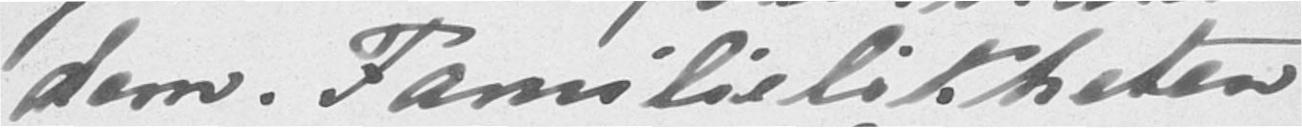dem. Familielikheten
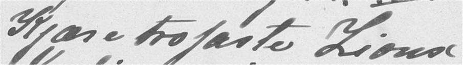Kjære trofaste Lious
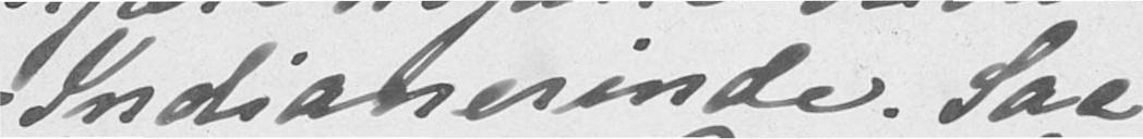-Indianerinde. Saa
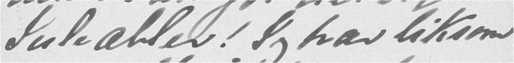Juleabler. Jeg har liksom
